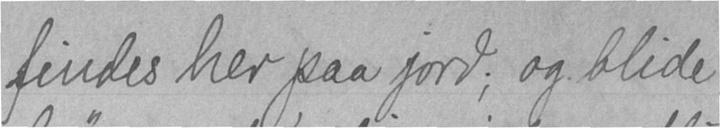findes her paa jord; og blide
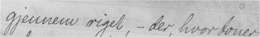gjennem riget, - der, hvor toner
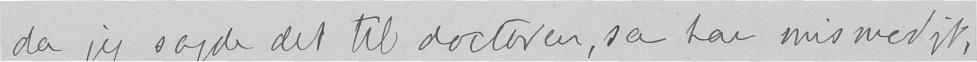da jeg sagde det til doctoren, sa han mismodigt,
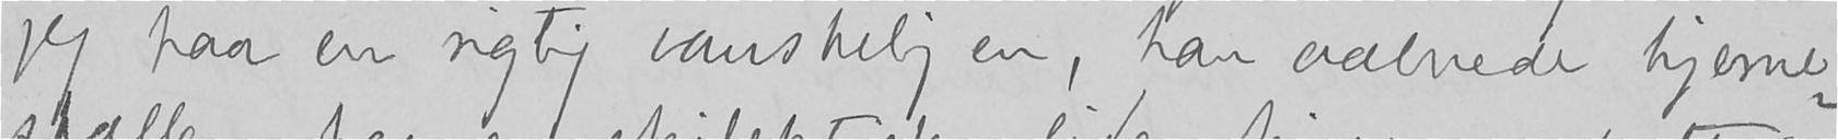jeg paa en rigtig vanskelig en, han aabnede hjerne-
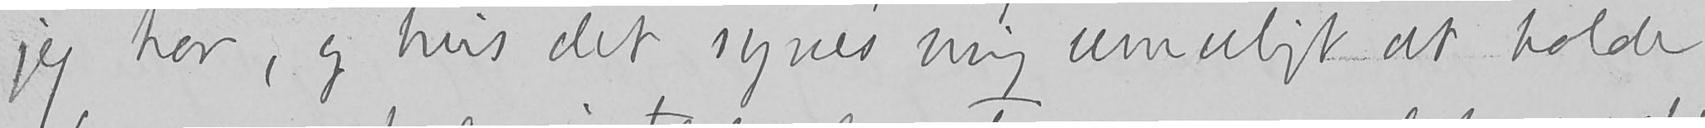jeg har, og hvis det synes mig umuligt at holde
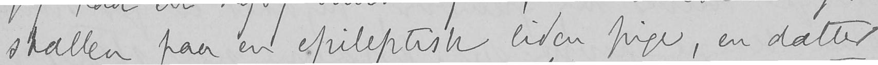skallen paa en epileptisk liden pige, en datter
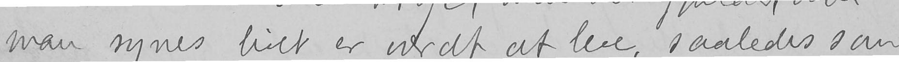man synes livet er værdt at leve, saaledes som
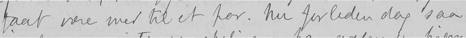faat være med til et par. Nu forleden dag saa
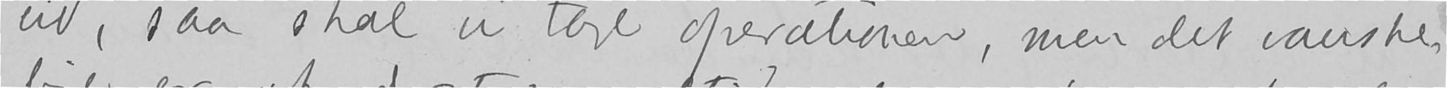ud, saa skal vi tage operationen, men det vanske-
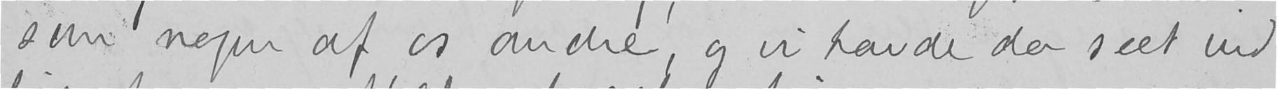som nogen af os andre, og vi havde da seet ind
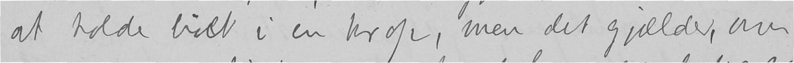at holde livet i en krop, men det gjælder, om
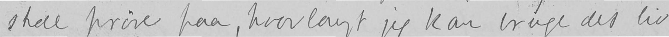skal prøve paa, hvorlangt jeg kan bruge det liv
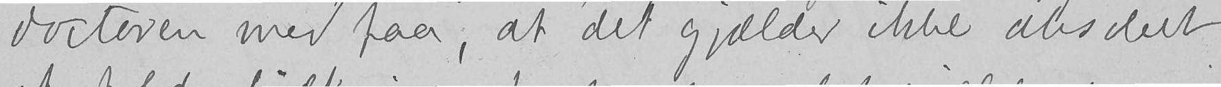doctoren med paa, at det gjælder ikke absolut
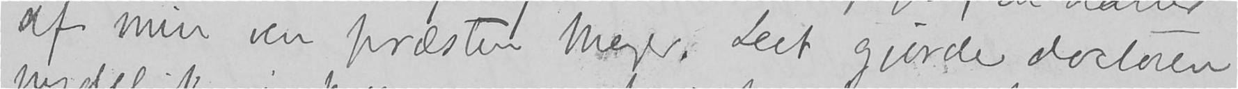af min ven præsten Meyer. Det gjorde doctoren
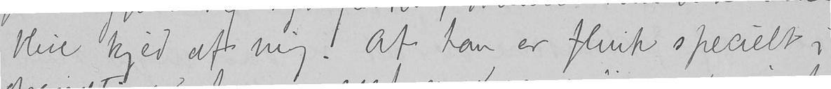blive kjed af mig. At han er flink specielt i
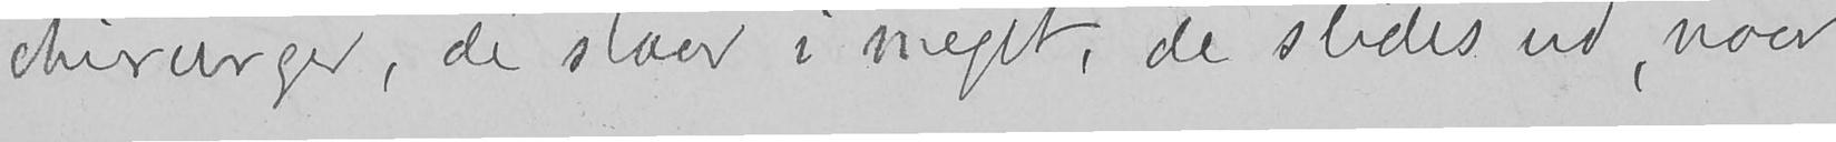chirurger, de staar i meget, de slides ud, naar
